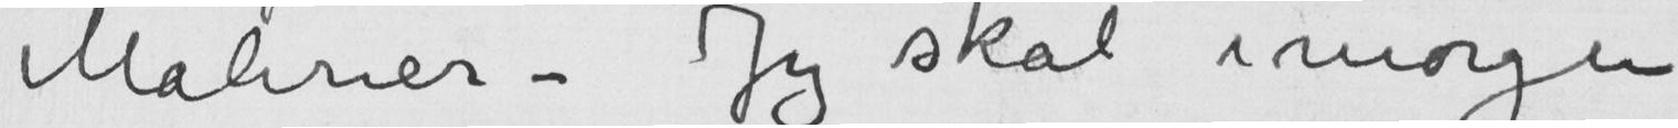Malerier – Jeg skal imorgen
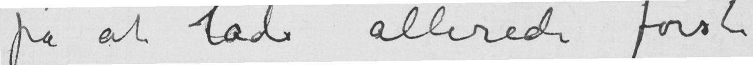på at lade allerede første
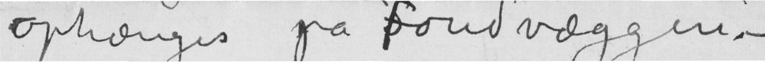ophænges på Fondvæggen? –
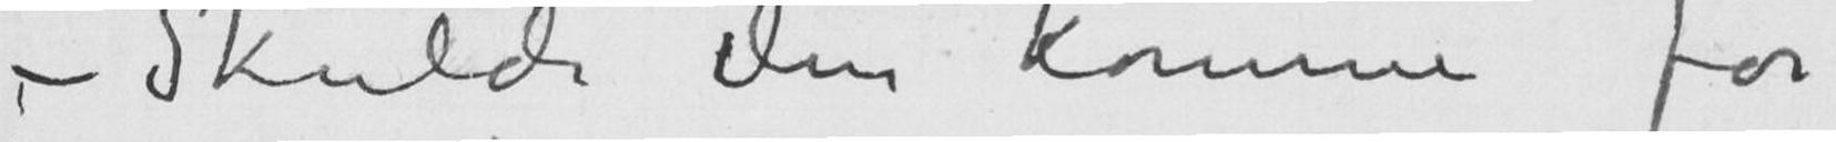– Skulde den komme for
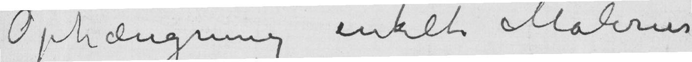Ophængning enkelte Malerier
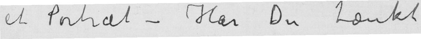et Portræt – Har Du tænkt
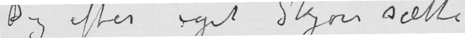Dig efter eget Skjøn sætte
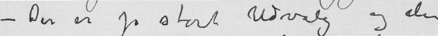– Der er jo stort Udvalg og den
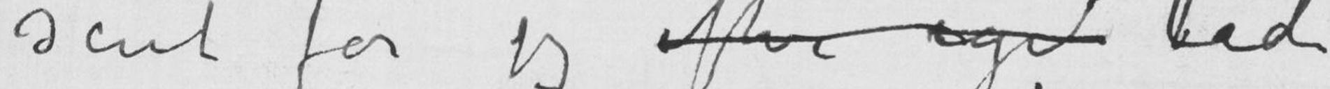sent får jeg efter eget lade
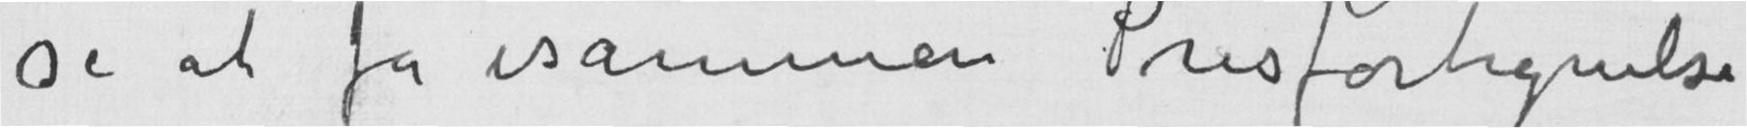se at få sammen Prisfortegnelse
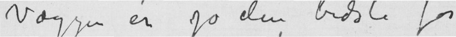Væggen er jo den bedste for
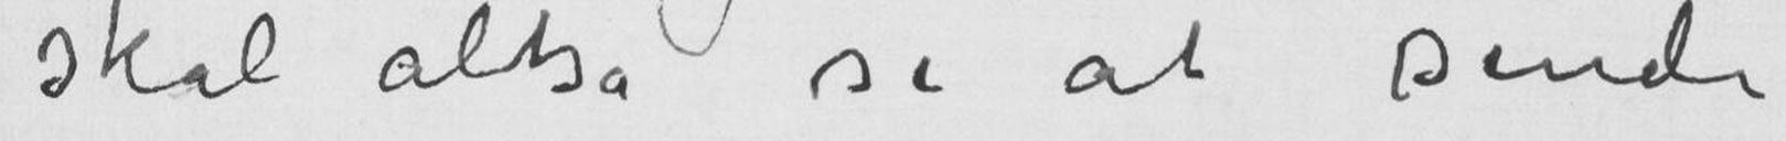skal altså se at sende
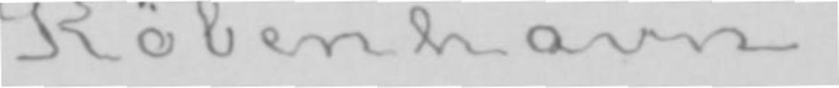København.
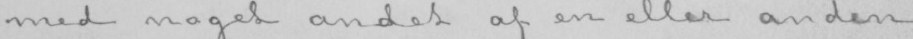med noget andet af en eller anden
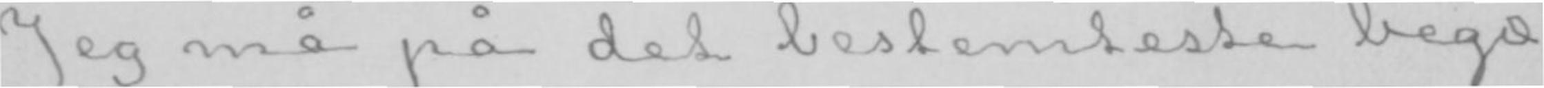Jeg må på det bestemteste begæ-
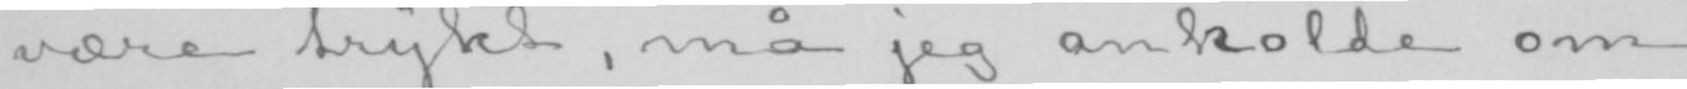være trykt, må jeg anholde om
